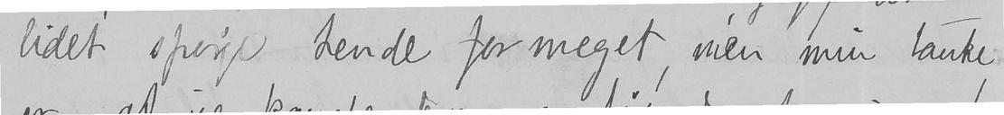lidet spørge hende for meget, men min tanke
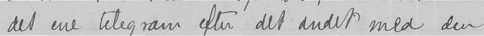det ene telegram efter det andet med den
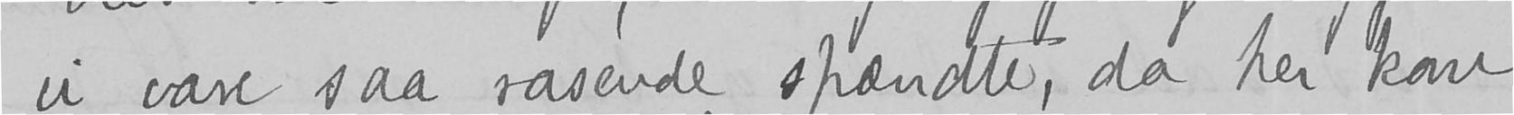vi vare saa rasende spændte, da her kom
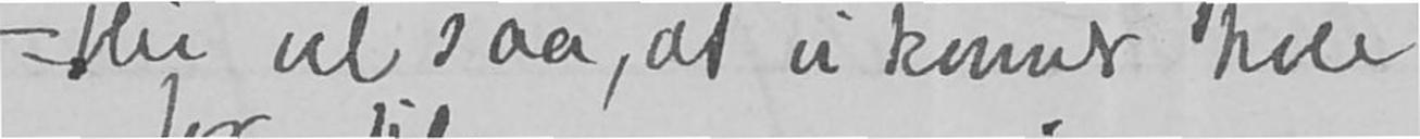blir vel saa, at vi kommer hele
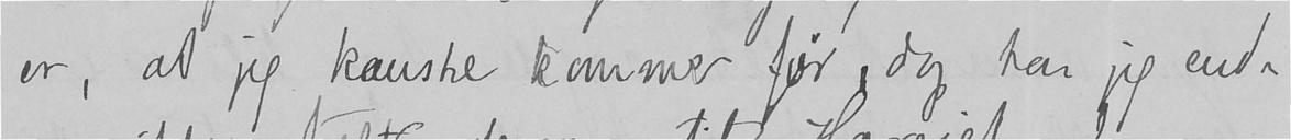er, at jeg kanske kommer før, dog har jeg end-
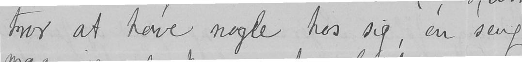tror at have nogle hos sig, en seng
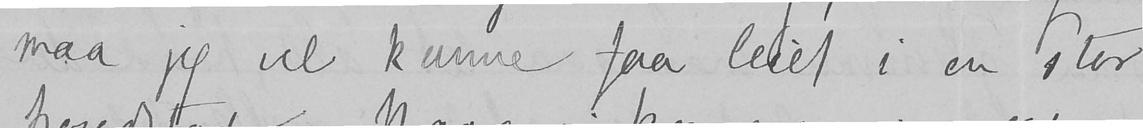maa jeg vel kunne faa leiet i en stor
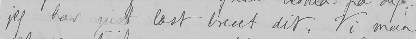jeg har just læst brevet dit. Vi maa
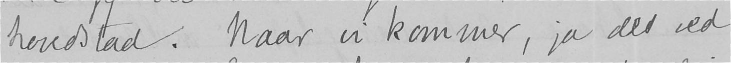hovedstad. Naar vi kommer, ja det ved
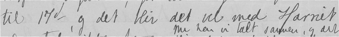til 17d, og det blir det vel med Harriet.
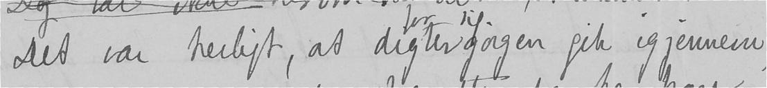Det var herligt, at digtergagen gik igjennem,
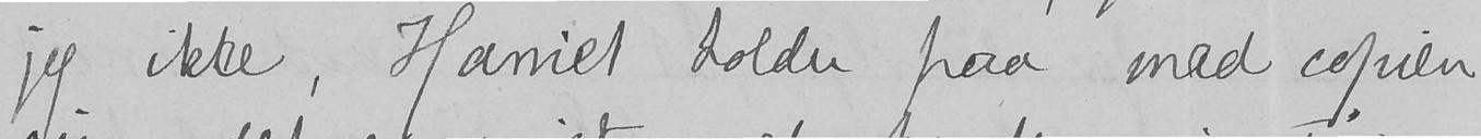jeg ikke, Harriet holder paa med copien
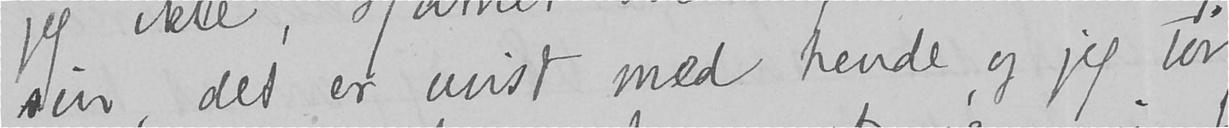sin, det er uvist med hende, og jeg tør
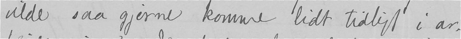vilde saa gjerne komme lidt tidligt i ar-
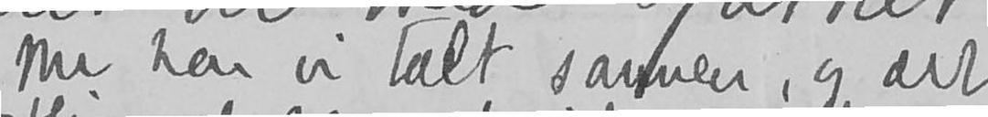Nu har vi talt sammen, og det
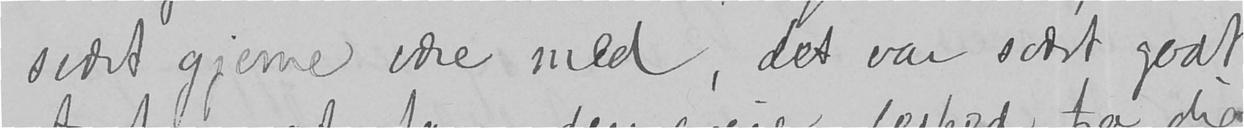svært gjerne være med, det var svært godt
